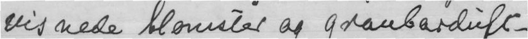visnede blomster og granbarduft -
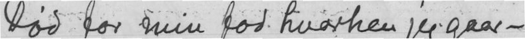Død for min fod hvorhen jeg gaar -
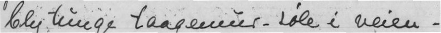blytunge taagemur - søle i veien -
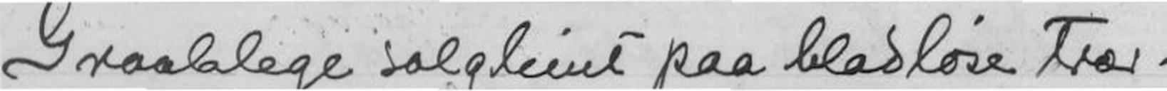Graablege solglimt paa bladløse Trær -
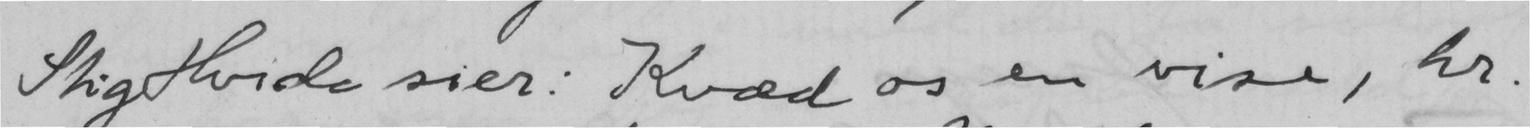Stig Hvide sier: Kvæd os en vise, hr.
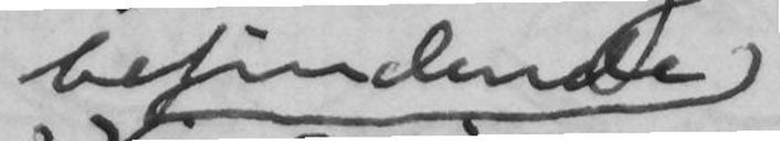befindende
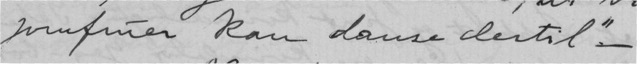jomfruer kan danse dertil" -
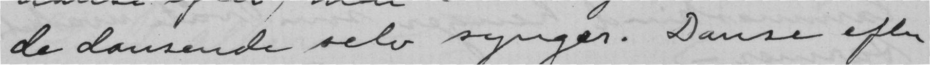de dansende selv synger. Danse efter
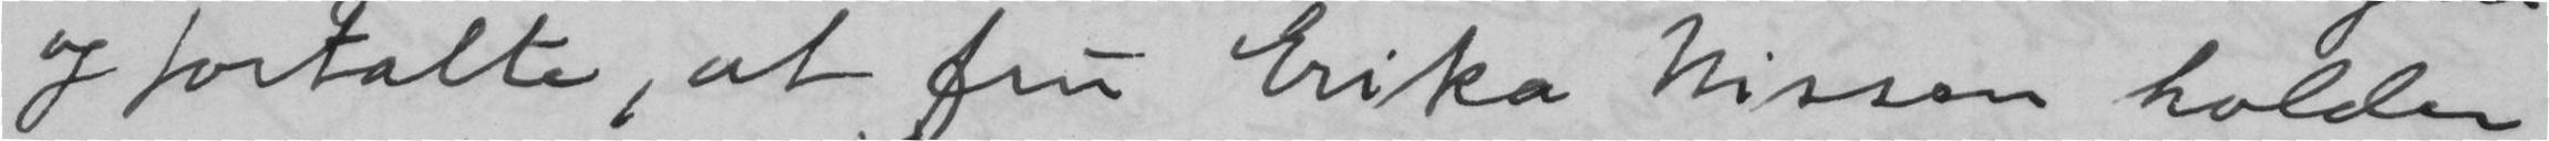og fortalte, at fru Erika Nissen holder
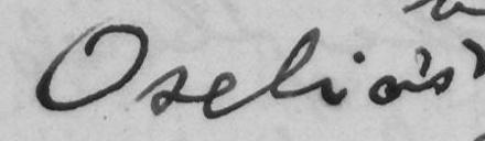Oselin's
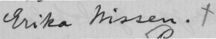Erika Nissen. x
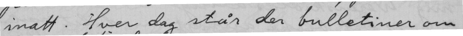inatt. Hver dag står der bulletiner om
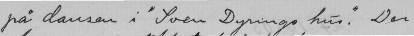på dansen i "Sven Dyrings hus". Der
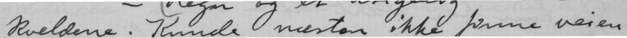kveldene. Kunde næsten ikke finne veien
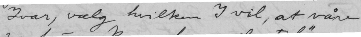Ivar, vælg hvilken I vil, at våre
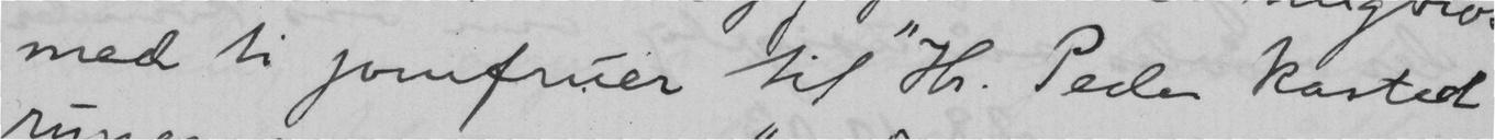med ti jomfruer til "Hr. Peder kasted
